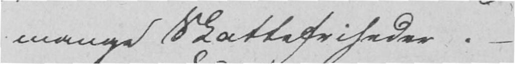mange Skattefriheder. -
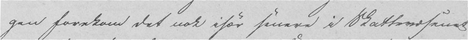gen forekom det nok især senere i Skattevæsenet
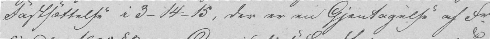Fastsættelse i 3-14-15, der er en Gjentagelse af Fr
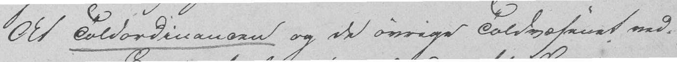At Toldordinancen og de øvrige Toldvæsenet ved-
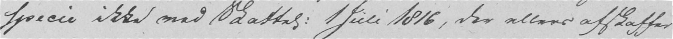specie ikke ved Skattels. 1 Juli 1816, der ellers afskaffer
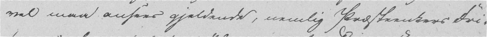vel maa ansees gjeldende, nemlig Præsteenkers Fri-
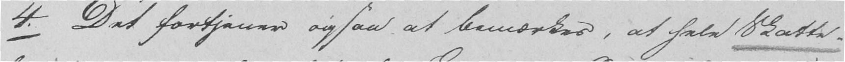4. Det fortjener ogsaa at bemærkes, at hele Skatte-
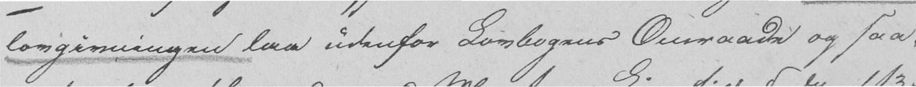lovgivningen laa udenfor Lovbogens Omraade og saa-
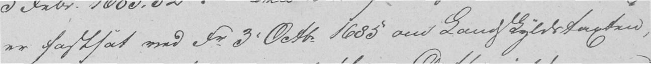er fastsat ved Fr 3. Octbr. 1685 om Landskyldstaxten,
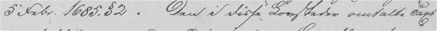5 Febr. 1685. § 2. Den i disse Lovsteder omtalte Taxt
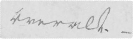overalt. -
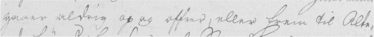gaaer aldrig op og offrer, eller frem til Alte-
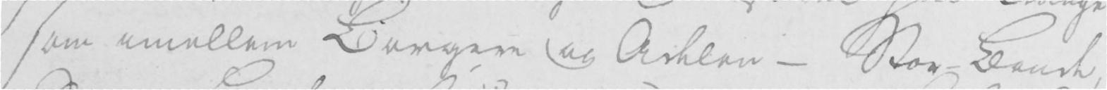som imellem Borgere og Adelen. Stor-Bonde,
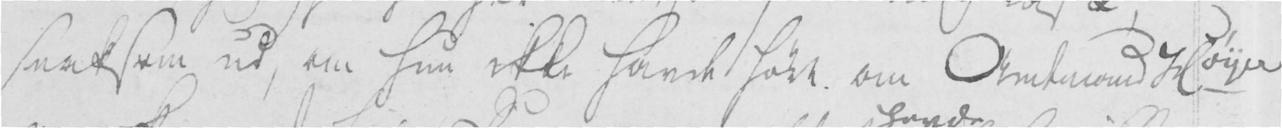snaksom ud, om hun ikke havde hørt om Amtmand Høyer
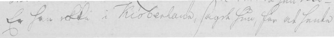Er han ikke i Kiøbenhavn, sagde hun, for at hente
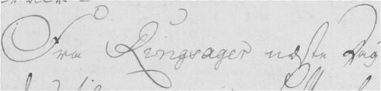Fra Ringsager næste Dag
